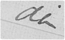din
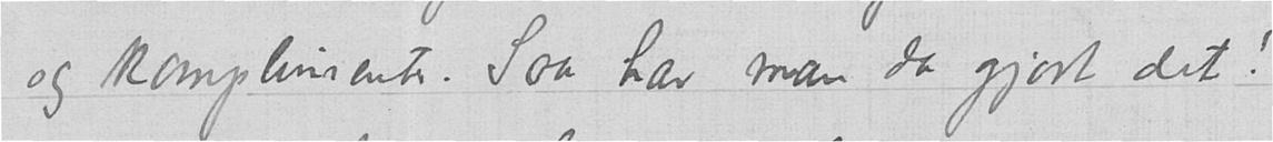og komplimenter. Saa har man da gjort det!
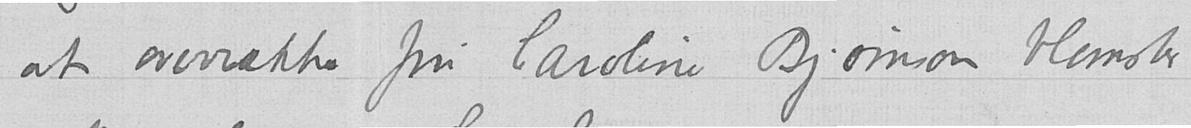at overrække fru Caroline Bjørnson blomster
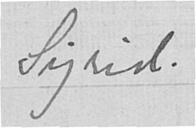Sigrid.
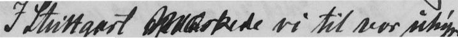I Stuttgart Mærkede vi til vor uhyre
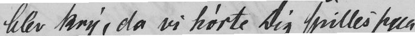blev kry, da vi hørte Dig spilles paa
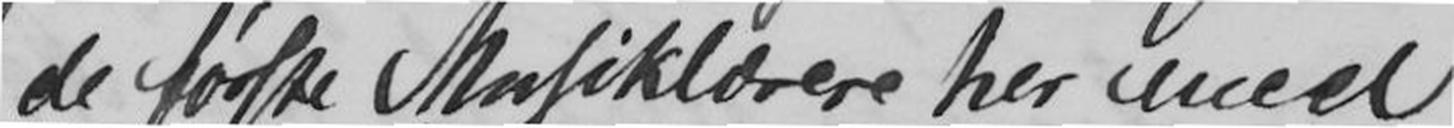de første Musiklærere her med
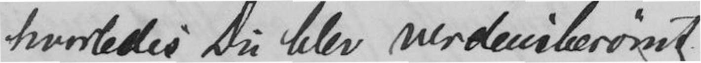hvorledes Du blev verdensberømt
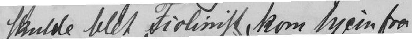skulde blit Fiolinist, kom hjem fra
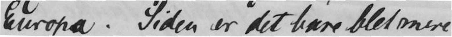Europa. Siden er det bare blit mere
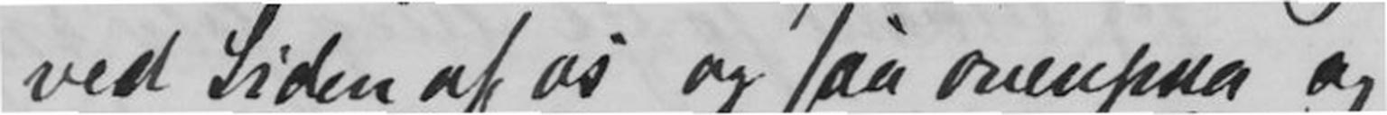ved Siden af os og saa ovenpaa og
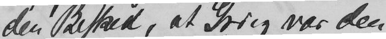den Besked, at Grieg var den
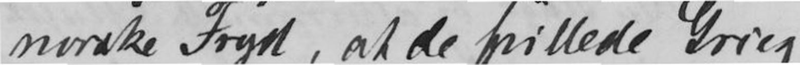norske Fryd, at de spillede Grieg
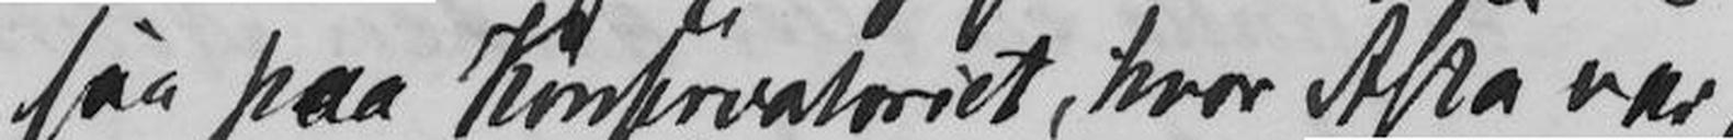saa paa Konservatoriet, hvor Asta var,
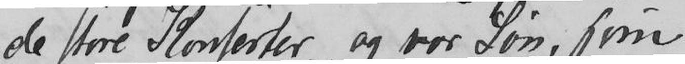de store Konserter og vor Søn, som
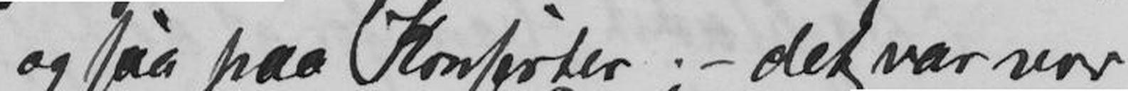og saa paa Konserter; - det var vor
